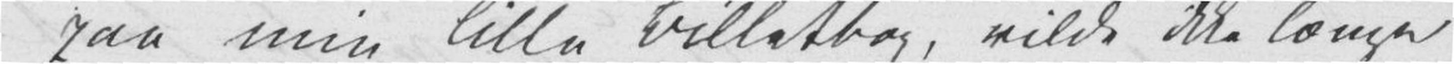paa min lille Billetbog, vilde ikke længe
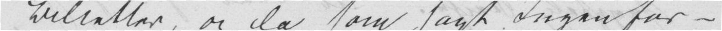Billetter, og da som sagt Ingen for¬
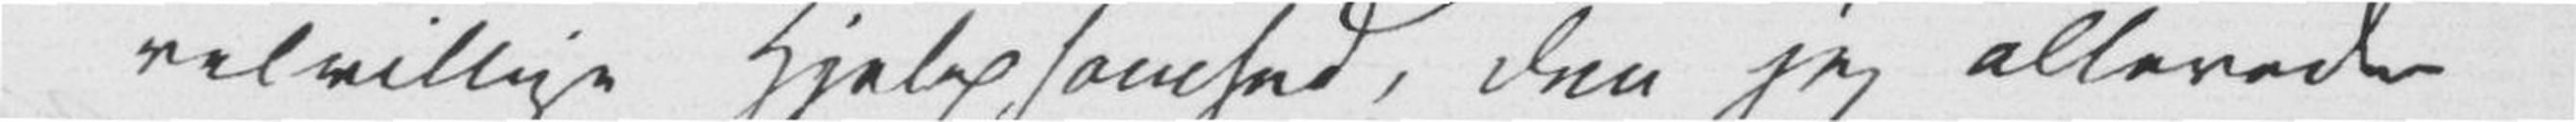velvillige Hjelpsomhed, den jeg allerede
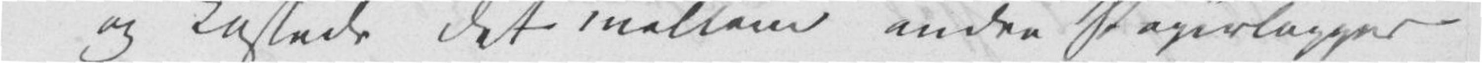og kastede det mellem andre Papirlapper
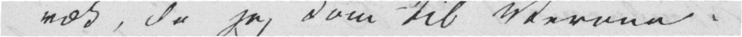væk, da jeg kom til Verona.
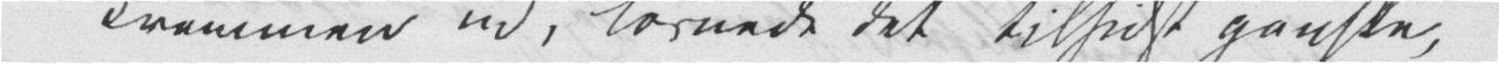kremmen ud, løsnede det tilsidst ganske,
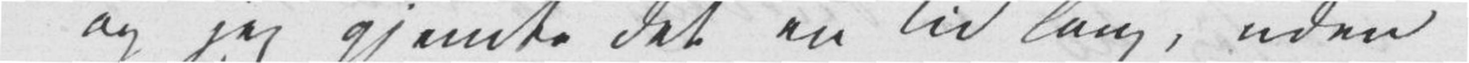og jeg gjemte det en Tid lang, uden
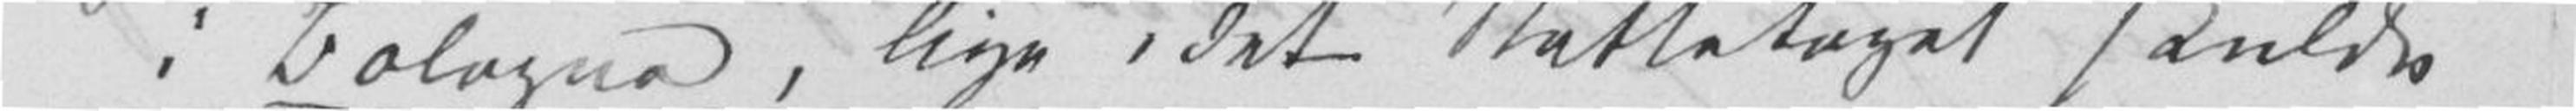i Bologna, lige idet Nattetoget skulde
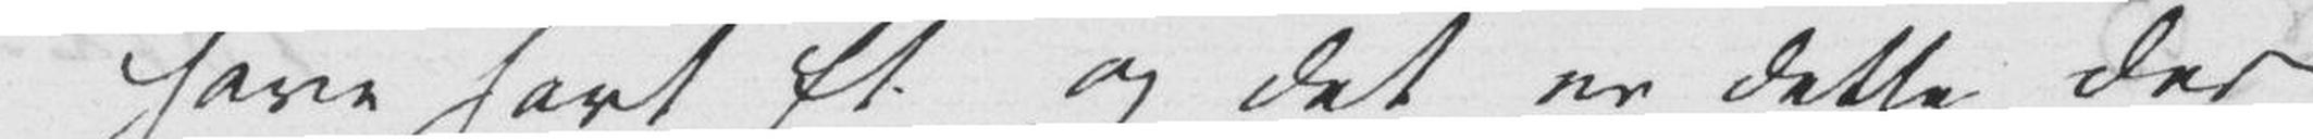have havt Et og det er dette der
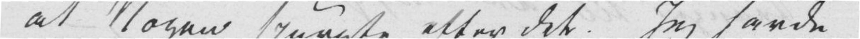at Nogen spurgte efter det. Jeg havde
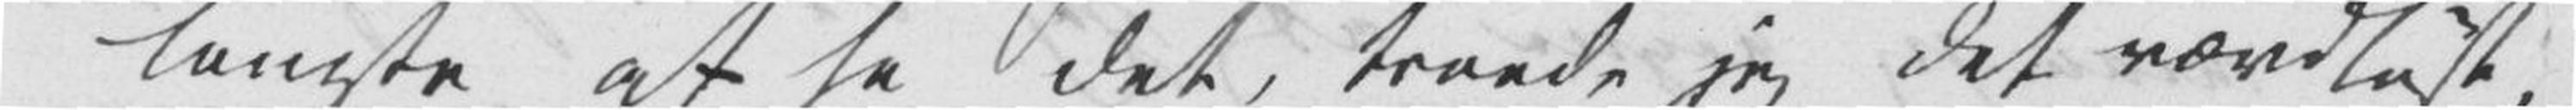langte at se det, troede jeg det værdløst,
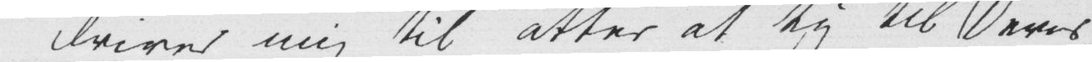driver mig til atter at ty til Deres
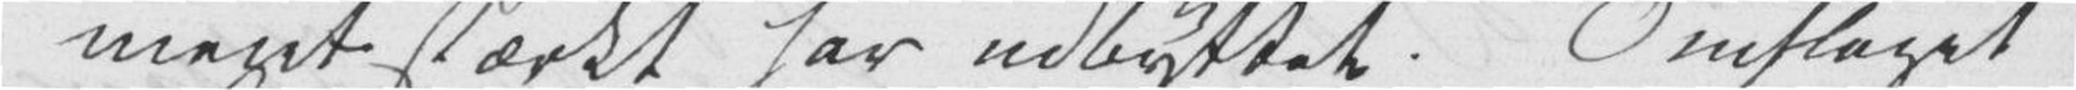meget stærkt har udbyttet. Omslaget
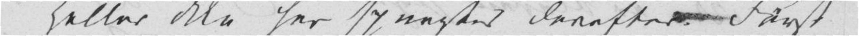Heller ikke her spurgtes derefter. Først
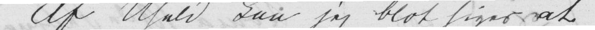Af Uheld kan jeg blot siges at
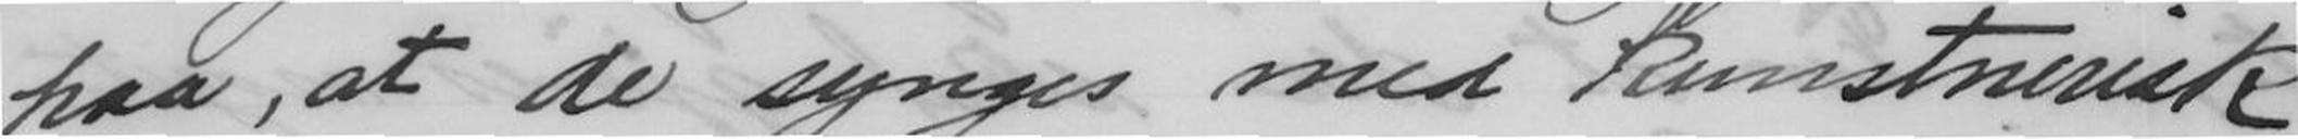paa, at de synges med kunstnerisk
